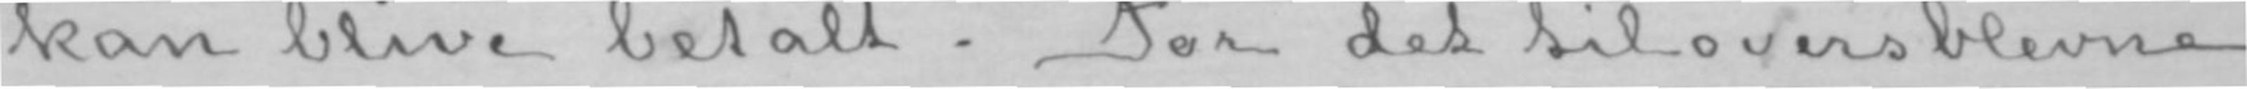kan blive betalt. For det tiloversblevne
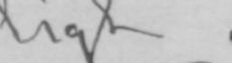ligt.
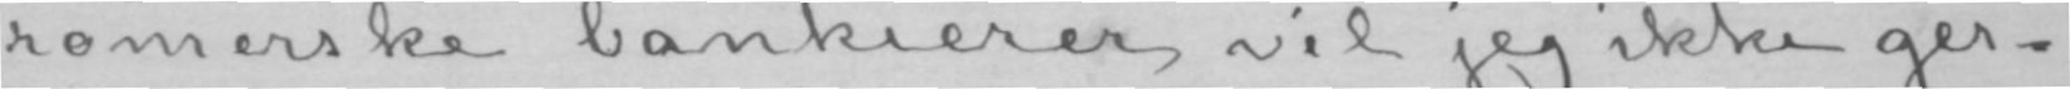romerske bankierer vil jeg ikke ger-
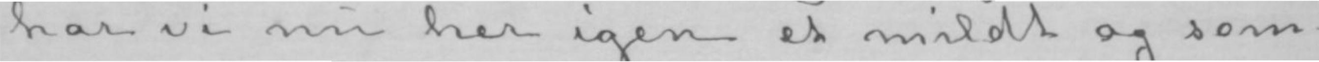har vi nu her igen et mildt og som-
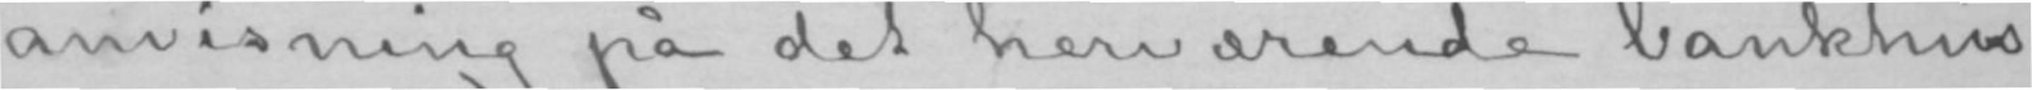anvisning på det herværende bankhus
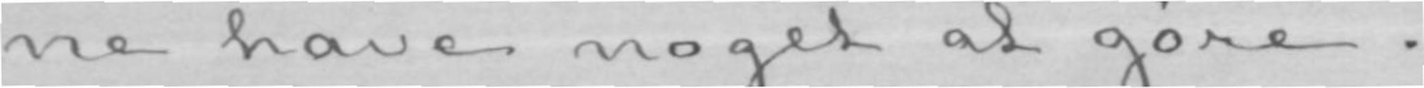ne have noget at gøre.
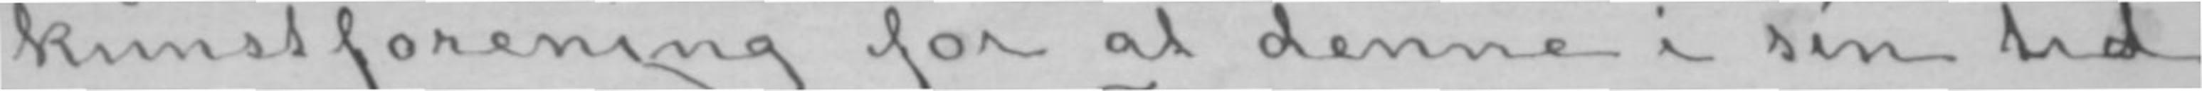kunstforening for at denne i sin tid
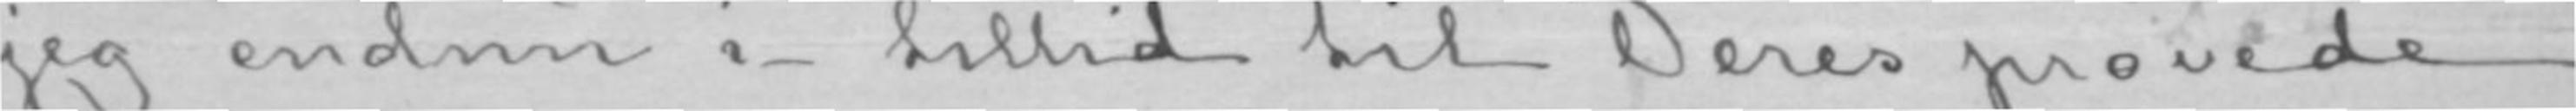jeg endnu i tillid til Deres prøvede
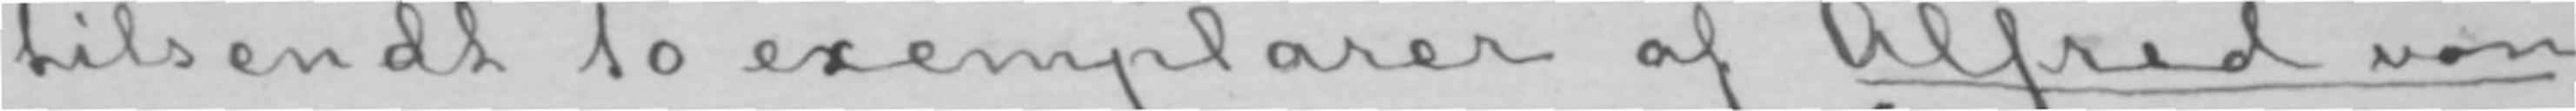tilsendt to exemplarer af Alfred von
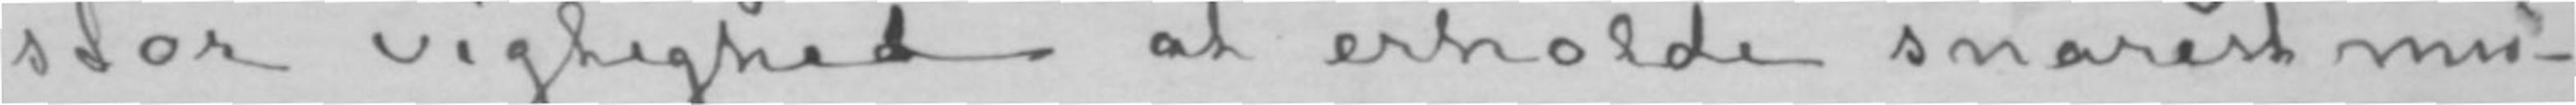stor vigtighed at erholde snarest mu-
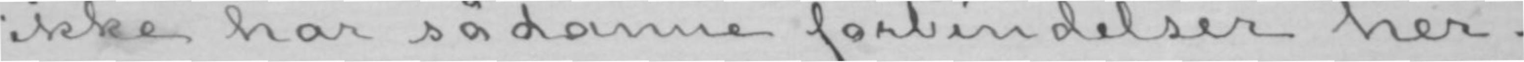ikke har sådanne forbindelser her-
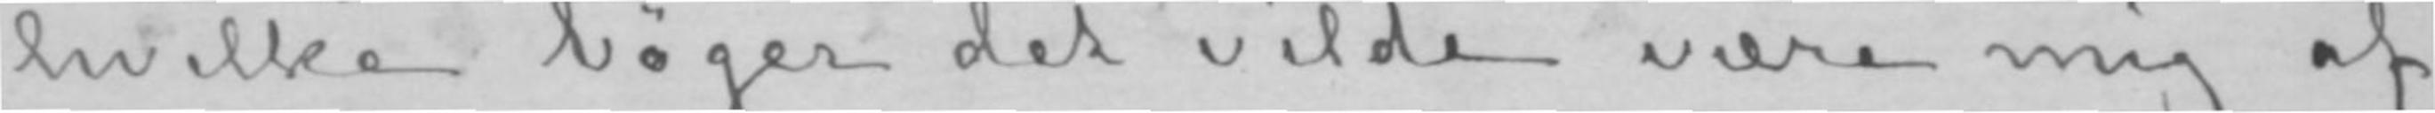hvilke bøger det vilde være mig af
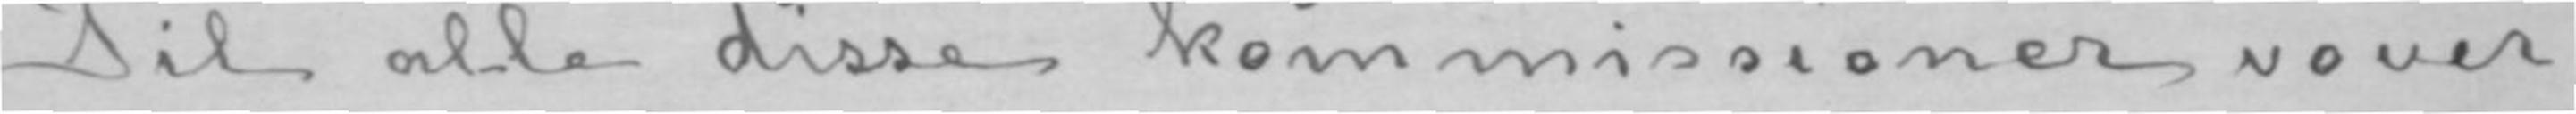Til alle disse kommissioner vover
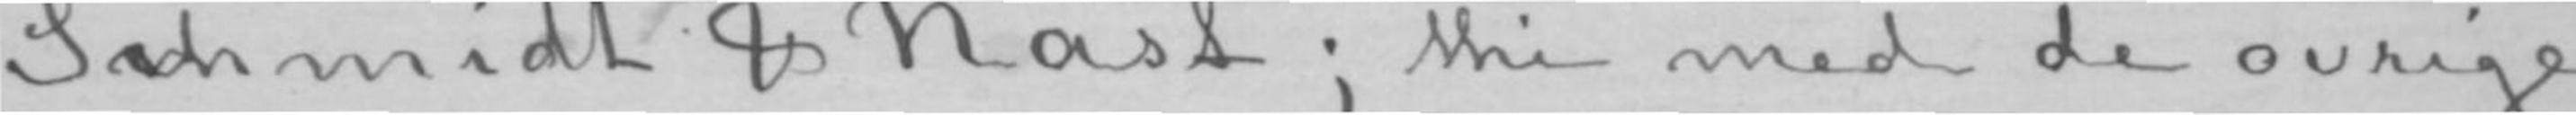Smchmidt & Nast; thi med de øvrige
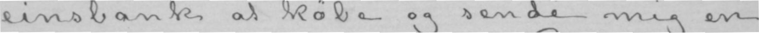einsbank at købe og sende mig en
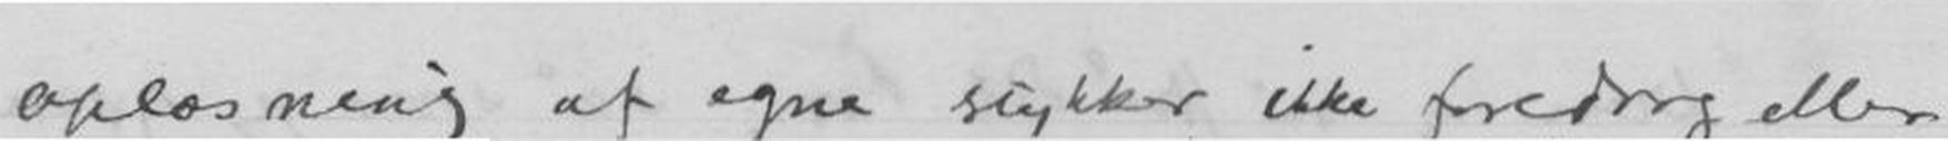oplæsning af egne stykker, ikke foredrag eller
